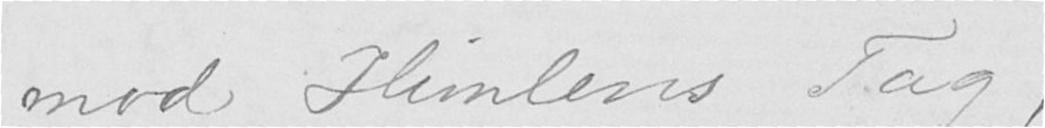mod Himlens Tag,
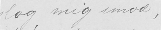slog mig imod,
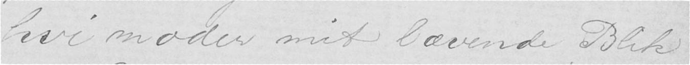hvi møder med bævende Blik
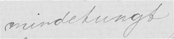mindetungt
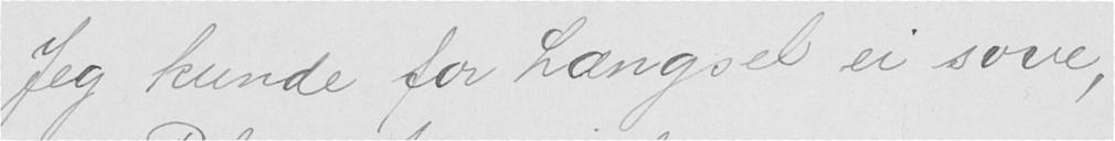Jeg kunde for Længsel ei sove,
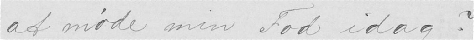at møde min Fod idag?
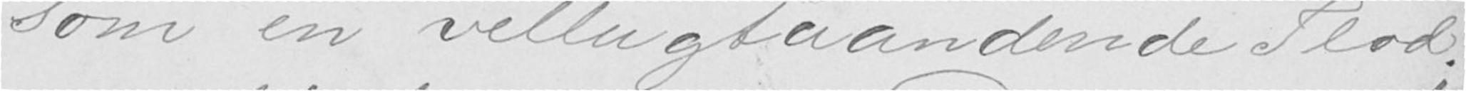som en vellugtaandende Flod;
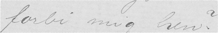forbi mig hen?
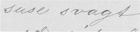suse svagt
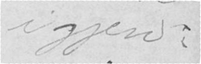igjen:
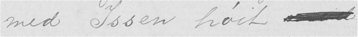med Issen høit
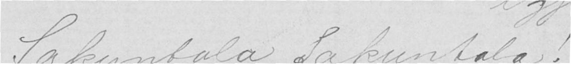Sakuntala, Sakuntala!
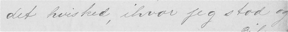det hvisked ihvor jeg stod og
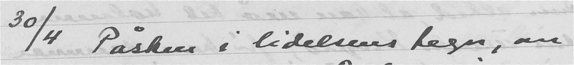30/4 Påsken i lidelsens tegn, om
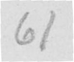61
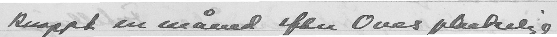knappt en måned efter Over plnkeligs
